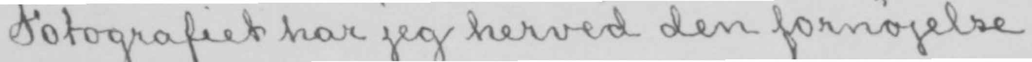Fotografiet har jeg herved den fornøjelse
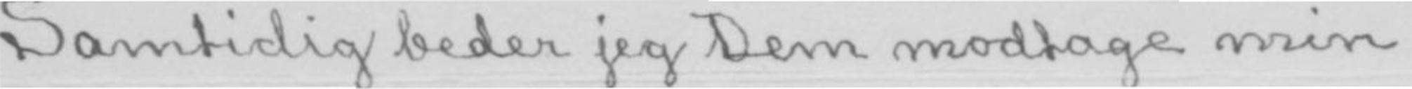Samtidigt beder jeg Dem modtage min
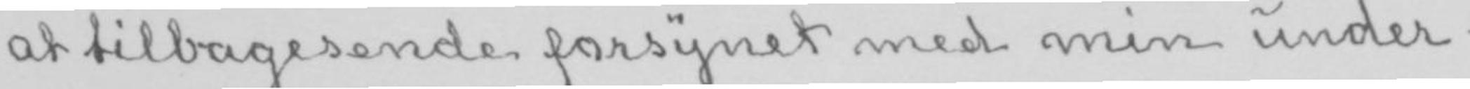at tilbagesende forsynet med min under-
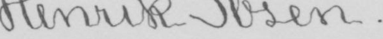Henrik Ibsen.
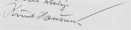Knut Hamsun.
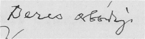Deres ærbødige
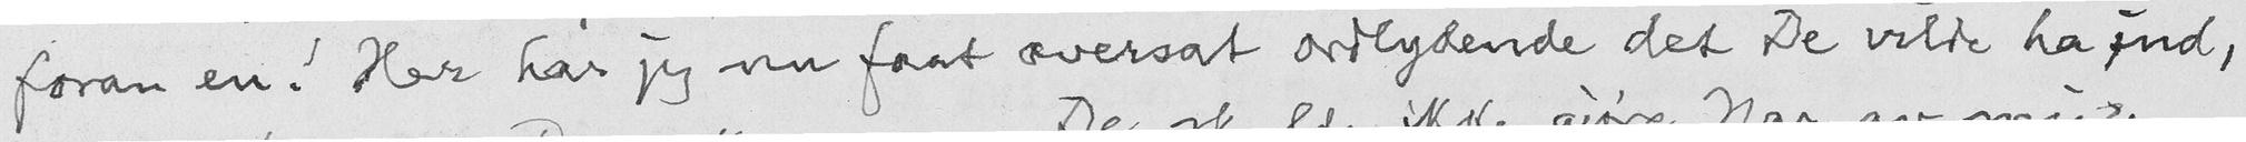foran en! Her har jeg nu faat oversat ordlydende det De vilde ha ind,
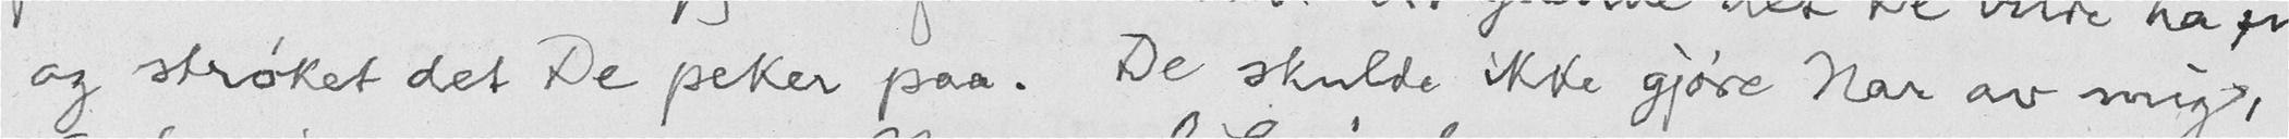og strøket det De peker paa. De skulde ikke gjøre Nar av mig,
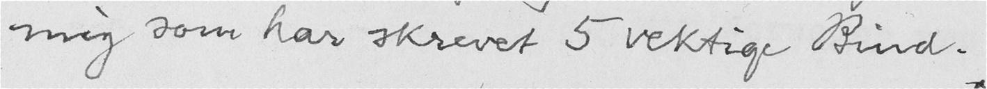mig som har skrevet 5 vektige Bind.
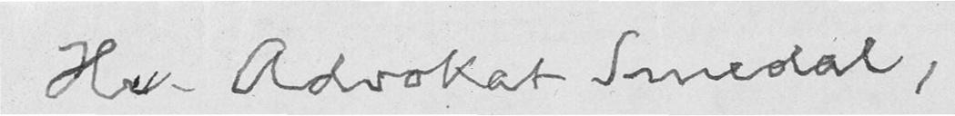Hr. Advokat Smedal,
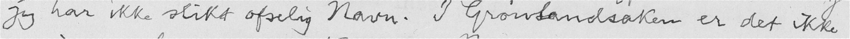jeg har ikke slikt ofselig Navn. I Grønlandsaken er det ikke
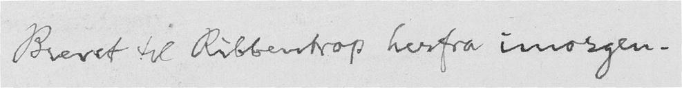Brevet til Ribbentrop herfra imorgen.
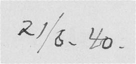21/6. 40.
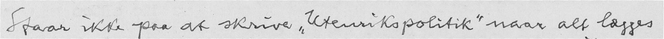Staar ikke paa at skrive "Utenrikspolitik" naar alt lægges
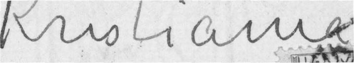Kristiania
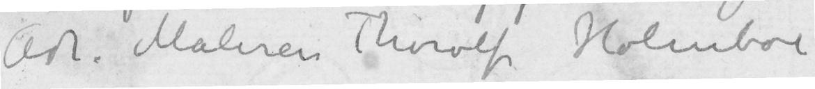Adr. Maleren Thorolf Holmboe
